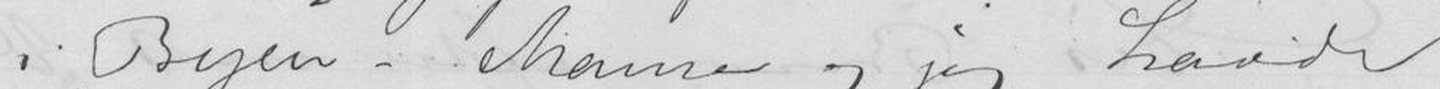i Byen - Mame og jeg havde
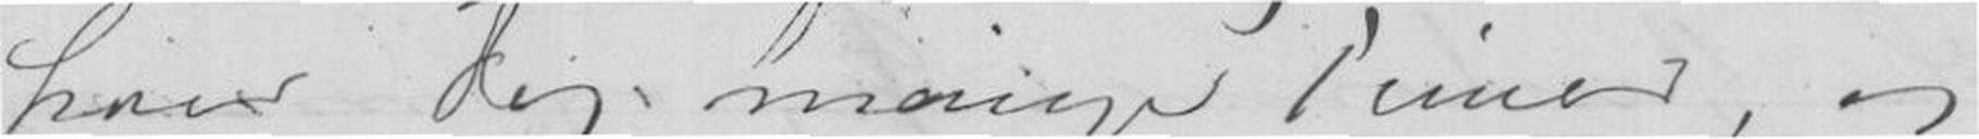hver Dag mange Timer, og
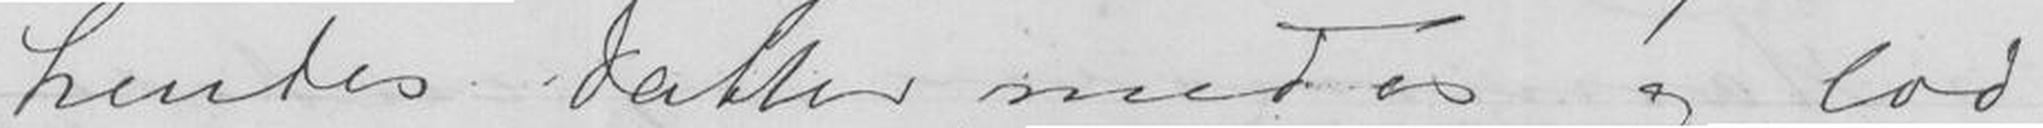hendes Datter med os og lod
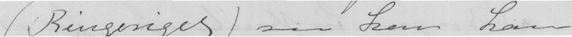(Ringeriget) saa kan han
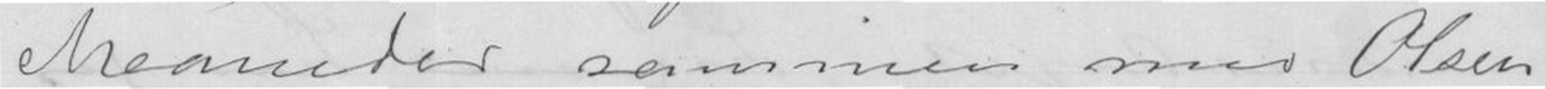Maaneder sammen med Olsen
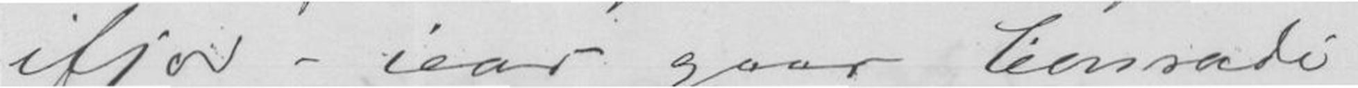ifjor - iaar gaar Conradi
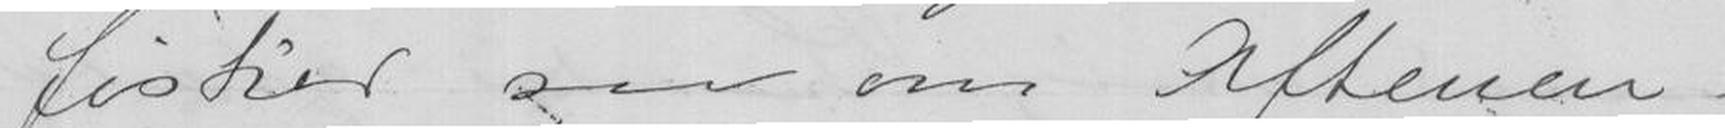fisker saa om Aftenen
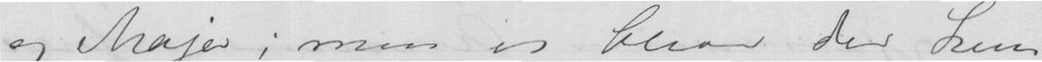og Maja; men vi bliver der kun
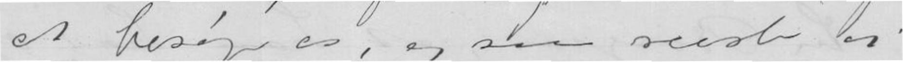at besøge os, og saa reiste vi
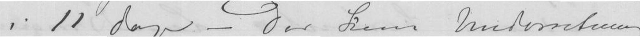i 11 Dage. Der kom Underretning
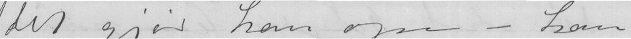det gjør han ogsaa - han
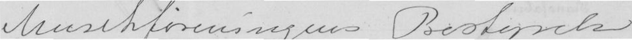Musikforeningens Bestyrelse
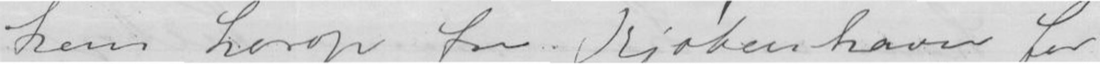kom herop fra Kjøbenhavn for
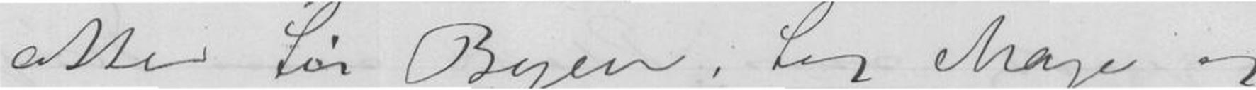atter til Byen, tog Maje og
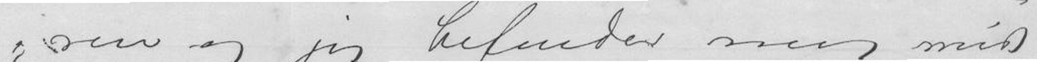ren og jeg befinder mig midt
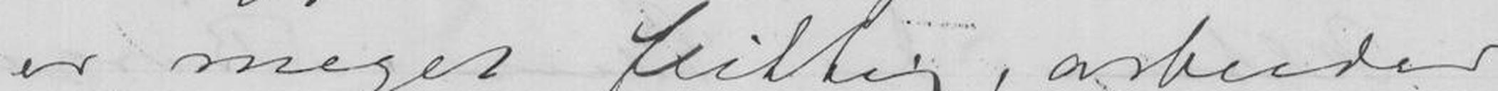er meget flittig, arbeider
